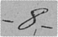- 8 -
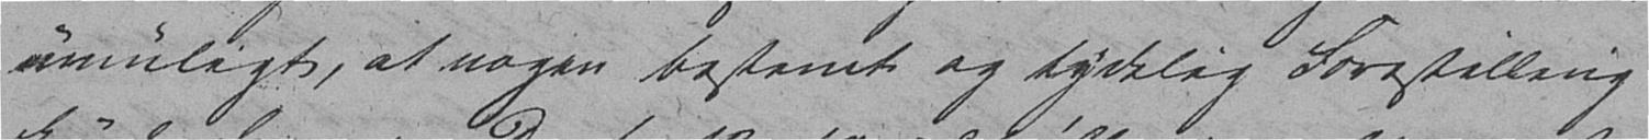umuligt, at nogen bestemt og tydelig Forestilling
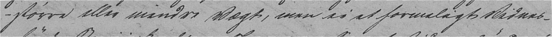større eller mindre Vægt, men ei et formeligt Vidnes-
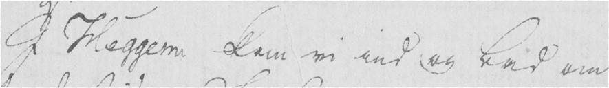I Heggem kom vi ind og bad om
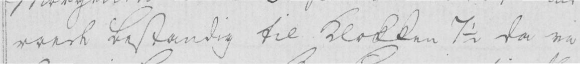roede bestandig til Klokken 7 1/2 da vi
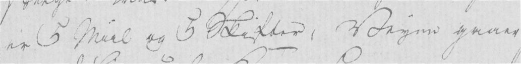er 5 Miil og 5 Skifter, Veyen gaaer
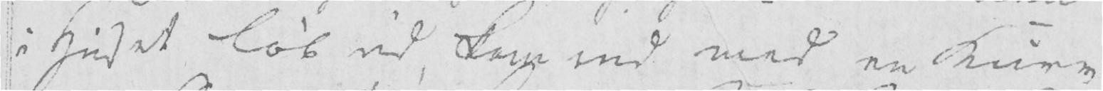i Huset løb ud, kom ind med en Kniv
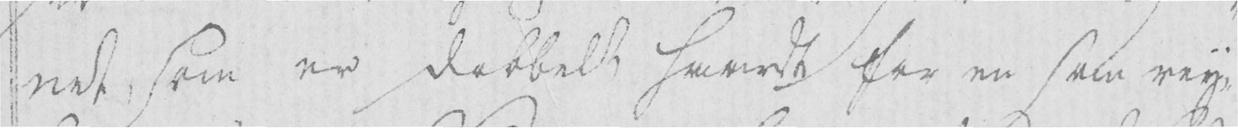net, som er dobbelt haardt for en som rey-
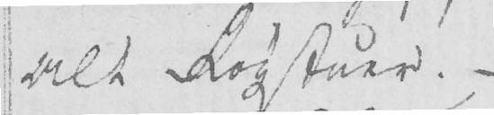alt Røgstuer. -
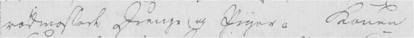rødmassede Drenge og Piger. Konen
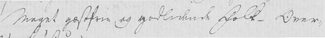Meget gæstfrie og godledende Folk - Over-
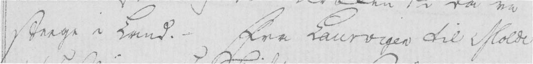steege i Land. Fra Laurvigen til Molde
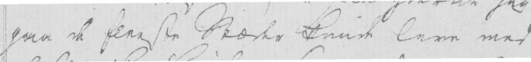paa de fleeste Stæder kunde leve med
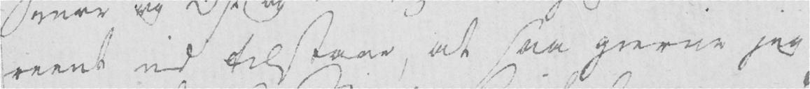reent ud tilstaae, at saa gierne jeg
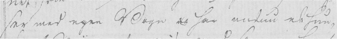ser med egen Vogn og har endnu et hun-
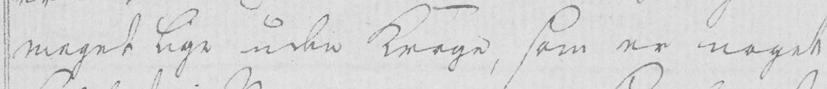meget lige uden Kroge, som er noget
